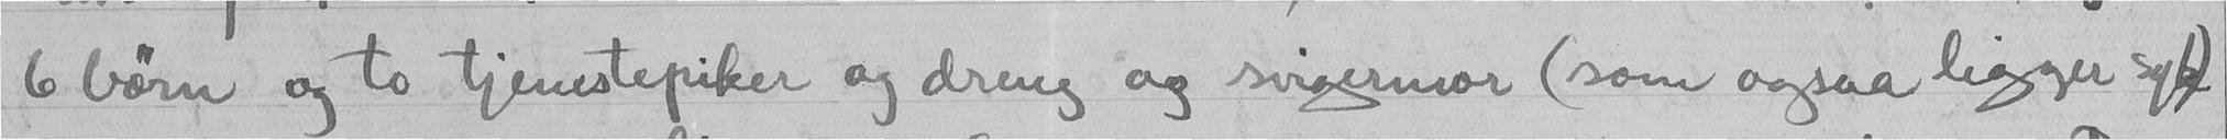6 børn og to tjenestepiker og dreng og svigermor (som ogsaa ligger syk)
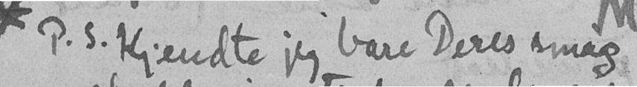* P.S. Kjendte jeg bare Deres smag
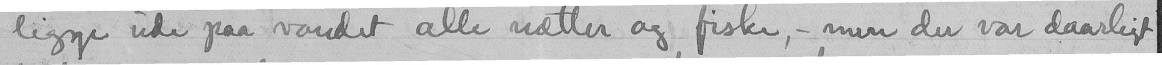ligge ude paa vandet alle nætter og fiske, - men der var daarligt
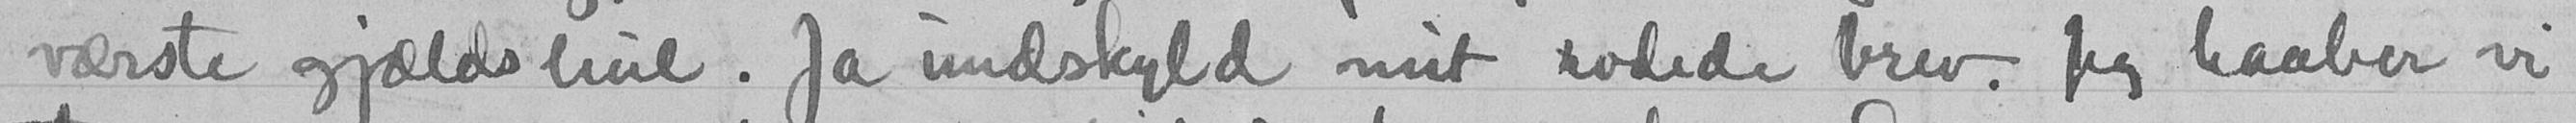værste gjældshul. Ja undskyld mit rodede brev. jeg haaber vi
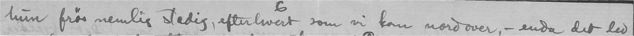hun frøs nemlig stadig, efterhvert som vi kom nordover, - enda det led
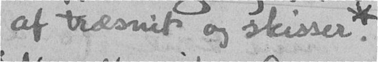af træsnit og skisser.*
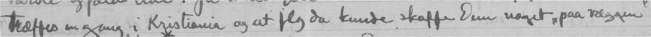træffes en gang i Kristiania og at jeg da kunde skaffe Dem noget "paa væggen"
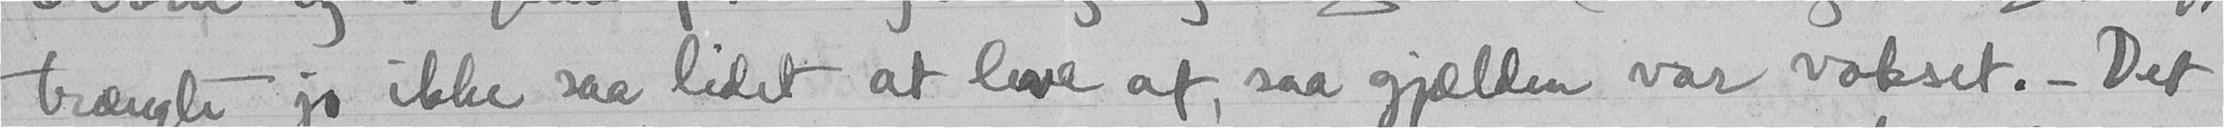trængte jo ikke saa lidet at leve af, saa gjælden var vokset. - Det
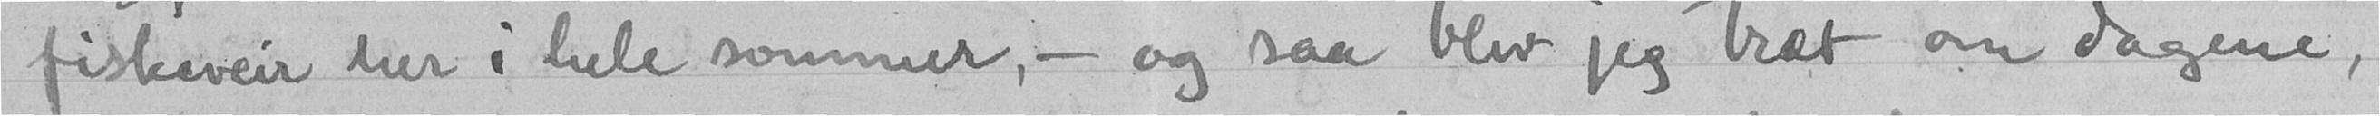fiskeveer her i hele sommer, - og saa bliv jeg træt om dagene,
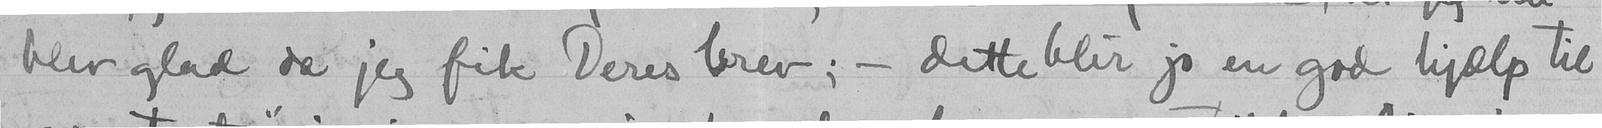blev glad da jeg fik Deres brev; - dette blir jo en god hjælp til
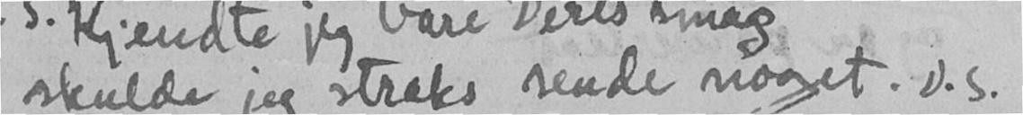skulde jeg straks sende noget. v.s.
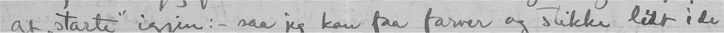at "starte" igjen: - saa jeg kan faa farver og stikke lidt i de
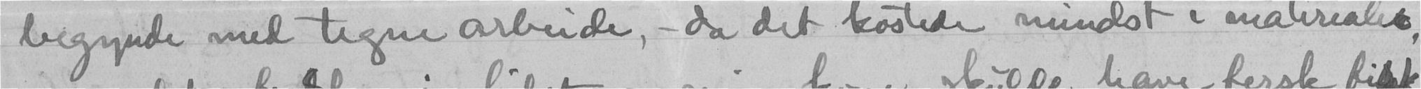begynde med tegne arbeide, - da det kostede mindst i materialet,
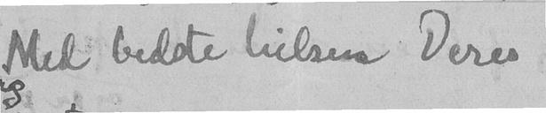Med bedste hilsen Deres.
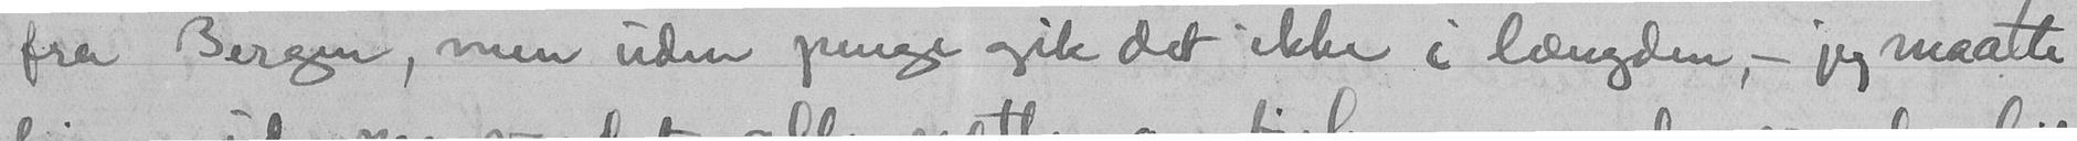fra Bergen, men uden penge gik det ikke i længden, - jeg maate
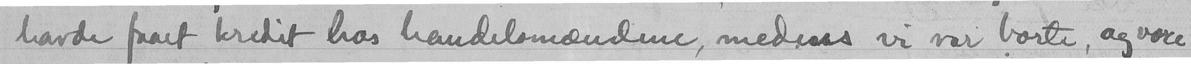havde faaet kredit hos handelsmændene, medens vi var borte, og vore
